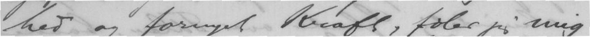hed og fornyet Kraft, føler jeg mig
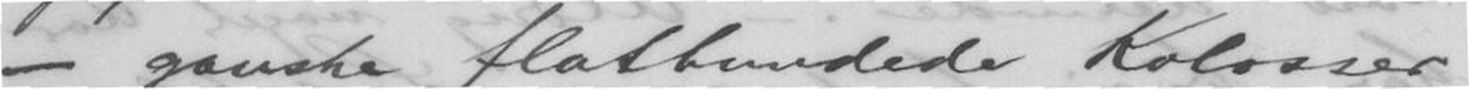- ganske flatbundede Kolosser -
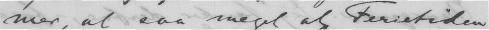mer, al saa meget at Ferietiden
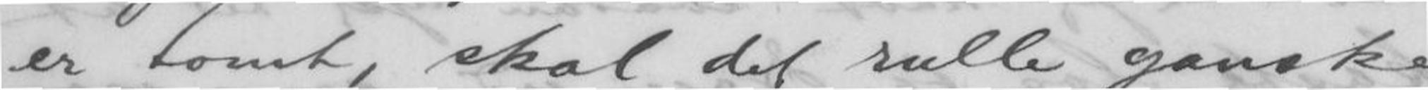er tomt, skal det rulle ganske
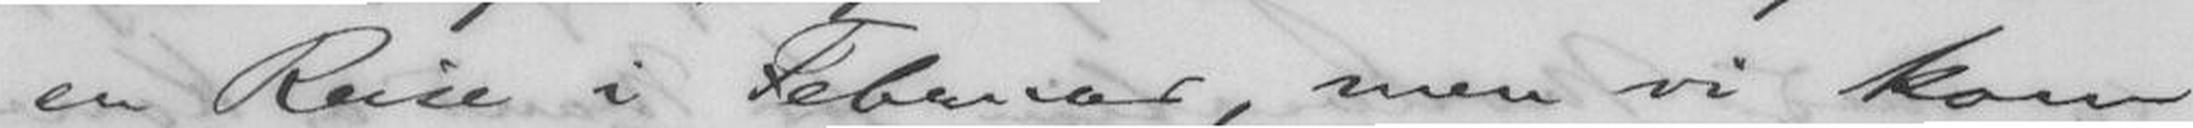en Reise i Februar, men vi kom
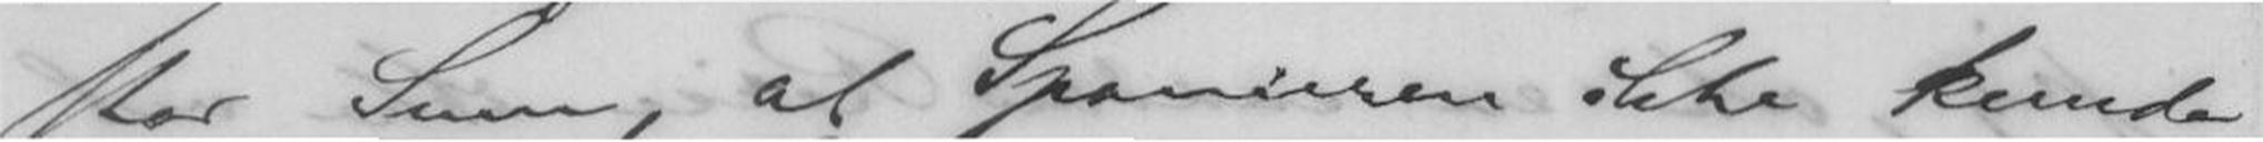stor Sum, at Spanieren ikke kunde
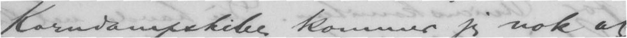Korndampskibe kommer jeg nok at
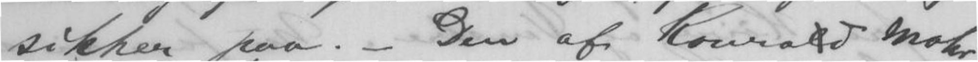sikker paa. - Den af Konrad "l" i Konrald Mohr
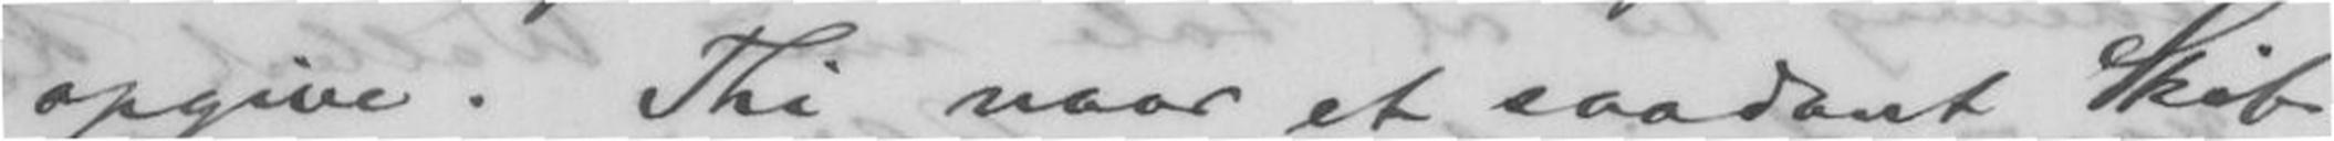opgive. Thi naar et saadant Skib
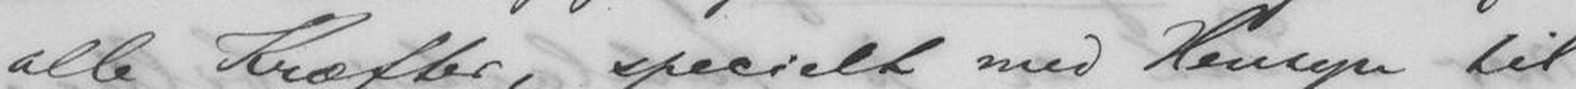alle Kræfter, specielt med Hensyn til
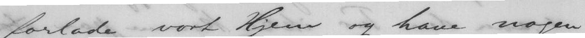forlade vort Hjem og have nogen
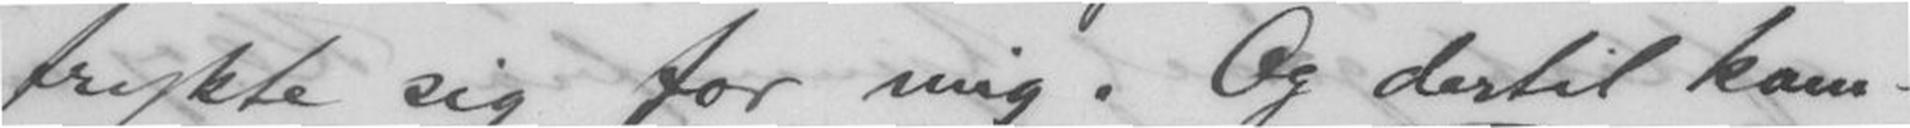trykte sig for mig. Og dertil kom-
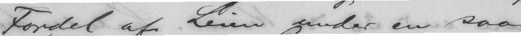Fordel af Leien under en saa
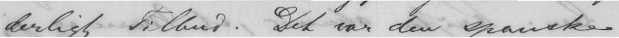derligt Tilbud. Det var den spanske
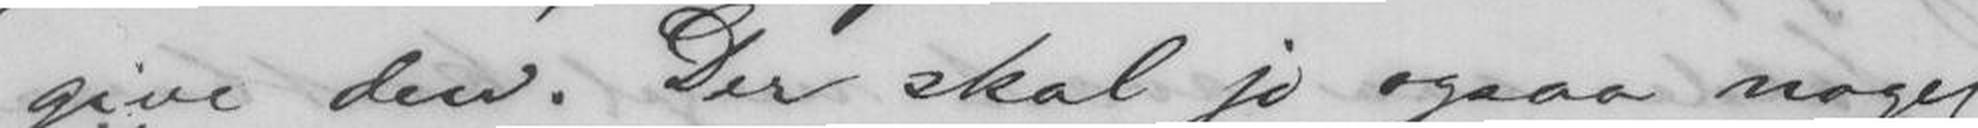give den. Der skal jo ogsaa noget
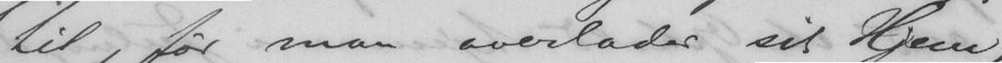til, før man overlader sit Hjem,
